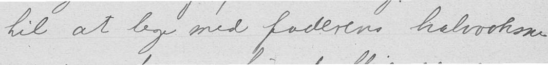til at lege med faderens halvvoksne.
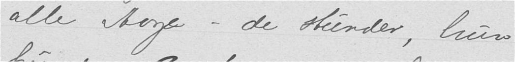alle Aage – de stunder, hun
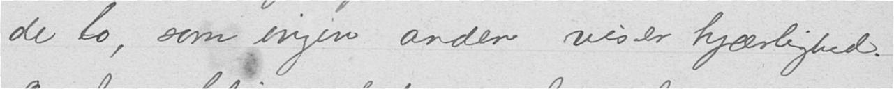de to, som ingen anden viser kjærlighed.
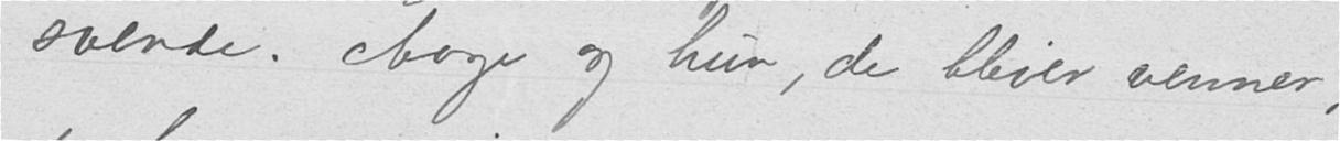svende. Aage og hun, de bliver venner,
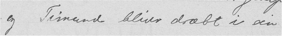og Timand bliver dræbt i sin
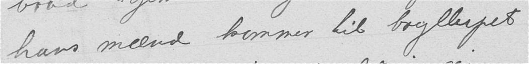hans mænd kommer til bryllupet
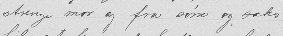strenge mor og fra søm og saks
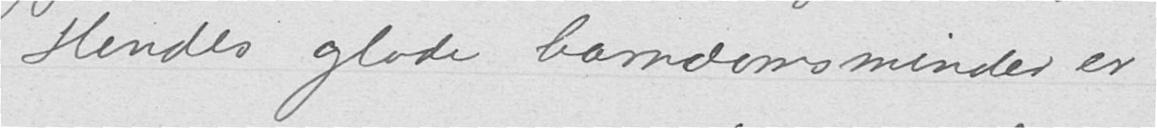Hendes glade bamdomsminder er
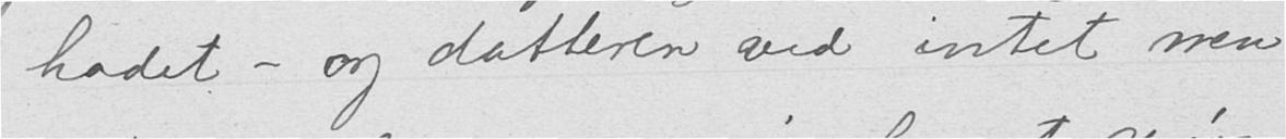hadet – og datteren ved intet men
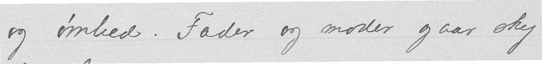og ømhed. Fader og moder gaar sky
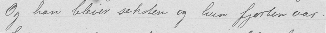Og han bliver seksten og hun fjorten aar.
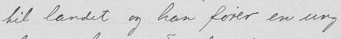til landet og han fører en ung
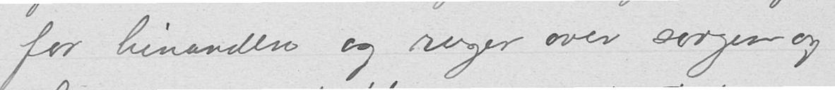for hinanden og ruger over sorgen og
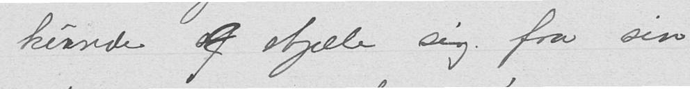kunde et stjæle sig fra sin
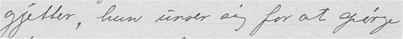gjetter, hun unser sig for at spørge.
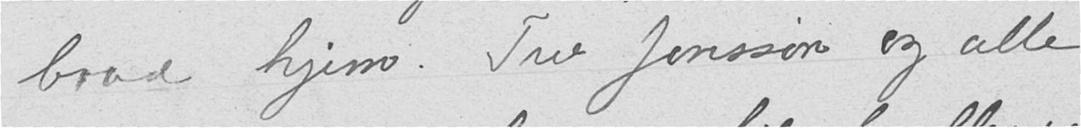brud hjem. Tue Jonssøn og alle
